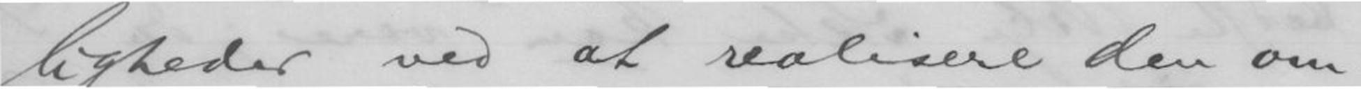ligheder ved at realisere den om-
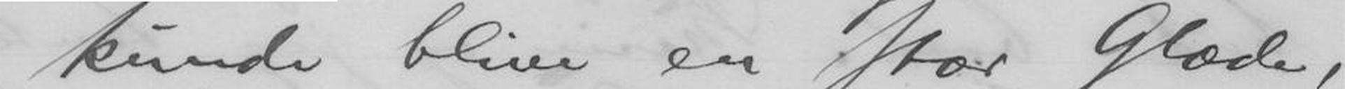kunde blive en stor Glæde!
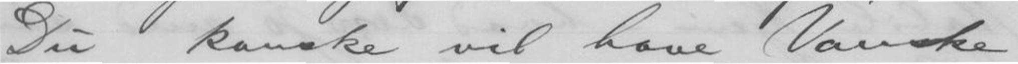Du kanske vil have Vanske-
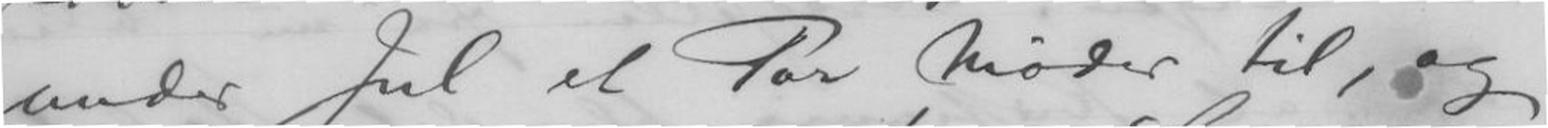under Jul et Par Møder til, og
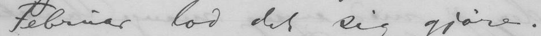Februar lod det sig gjøre.
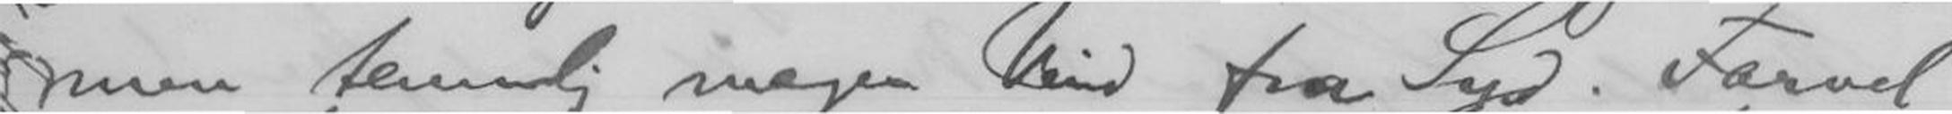men temmelig megen Vind fra Syd. Farvel
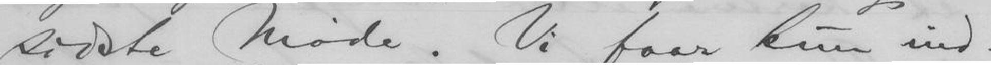sidste Møde. Vi faar kun ind-
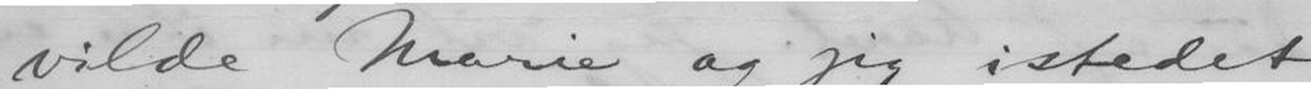vilde Marie og jeg istedet
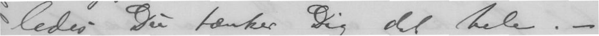ledes Du tænker Dig det hele. -
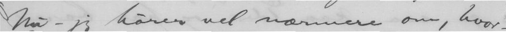Nu - jeg hører vel nærmere om, hvor-
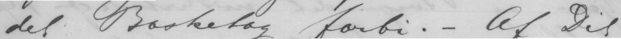det Basketag forbi. - Af Dit
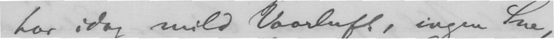har idag mild Vaarluft, ingen Sne,
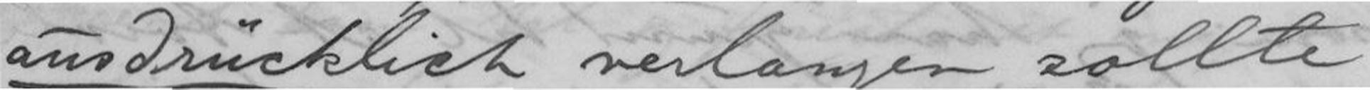ausdrücklich verlangen sollte,
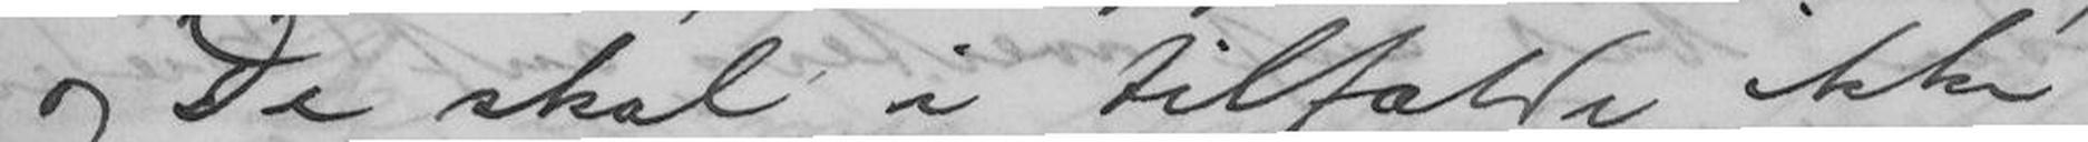og De skal i tilfælde ikke
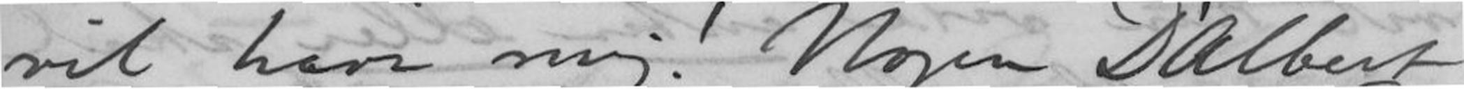vil have mig! Nogen D'Albert
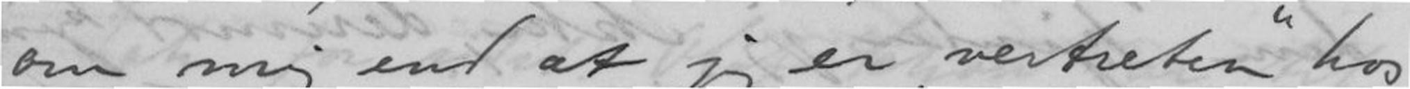om mig end at jeg er "vertreten" hos
